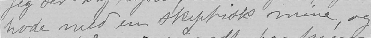hode med en skeptisk mine, og
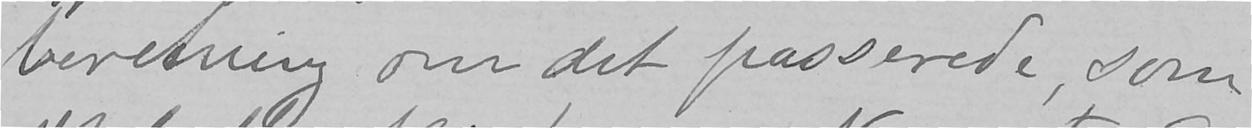beretning om det passerede, som
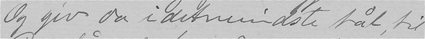Og giv da idetmindste tål, til
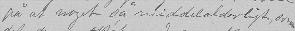på at noget så middelalderligt, som
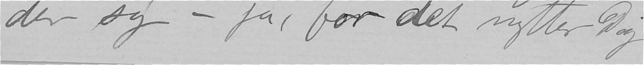der sig - ja, for det nytter Dig
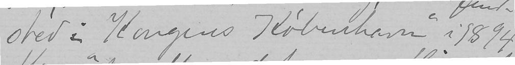sted i "Kongens København" i 1894
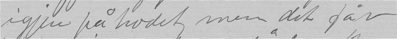igjen på hodet, men det får
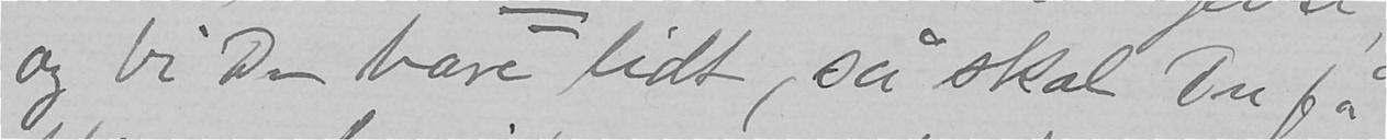og bi Du bare lidt, så skal Du få
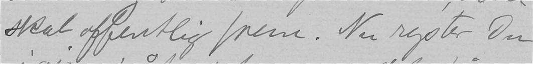skal offentlig frem. Nu ryster Du
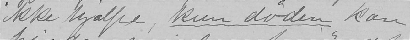ikke hjælpe, kun døden kan
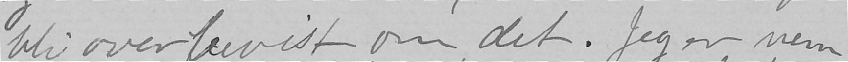bli overbevist om det. Jeg er nem-
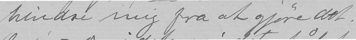hindre mig fra at gjøre det.
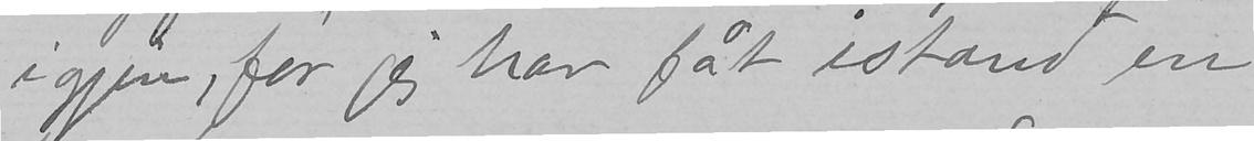igjen, før jeg har fåt istand en
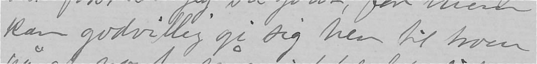kan godvillig gi sig hen til troen
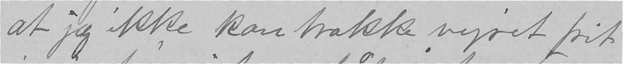at jeg ikke kan trække vejret frit
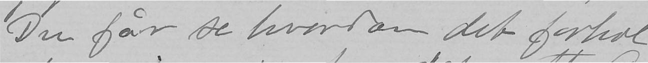Du får se hvordan det forhol
